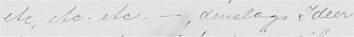etc. etc. etc. — denslags Ideer
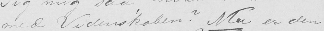med Videnskaben? Nu er den
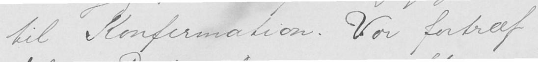til Konfirmation. Vor fortræf-
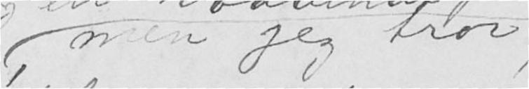men jeg tror,
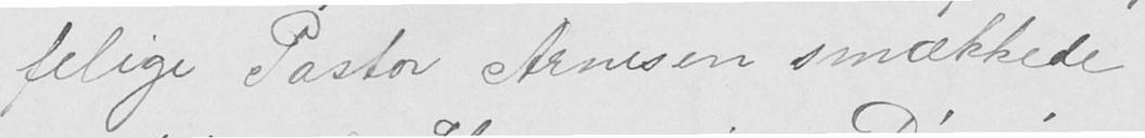felige Pastor Arnesen smækkede
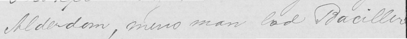Alderdom, mens man lod Baciller
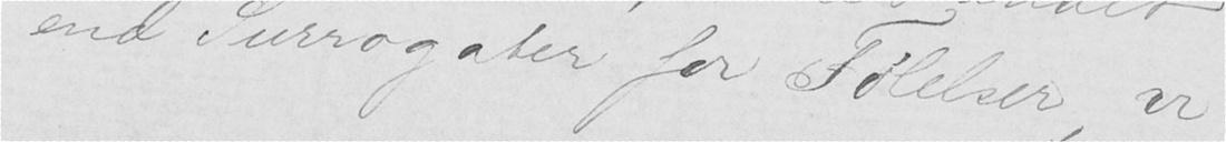end Surrogater for Følelser, vi
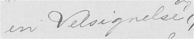en Velsignelse,
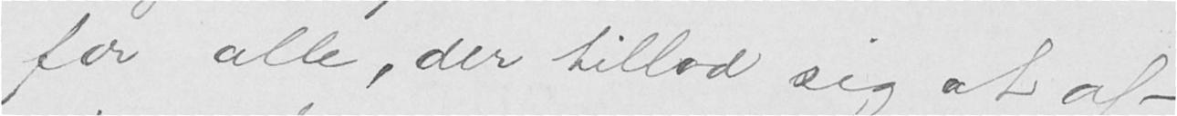for alle, der tillod sig at af-
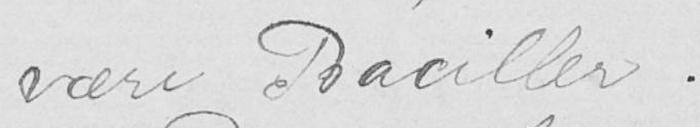være Baciller.
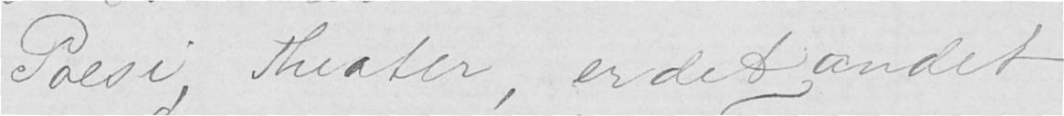Poesi, Theater, er det andet
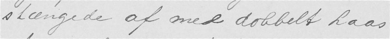stængede af med dobbelt Laas
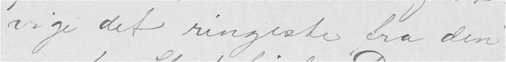vige det ringeste bra den
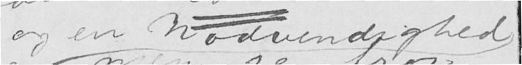og en Nødvendighed,
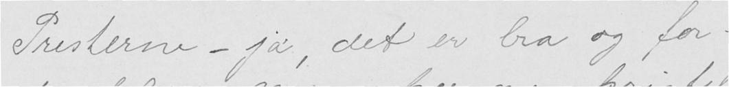Presterne - ja, det er bra og for-
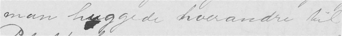man huggede hverandre til
